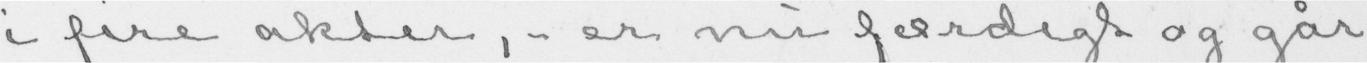i fire akter,- er nu færdigt og går
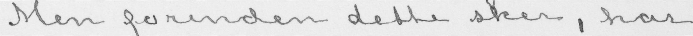Men forinden dette sker, har
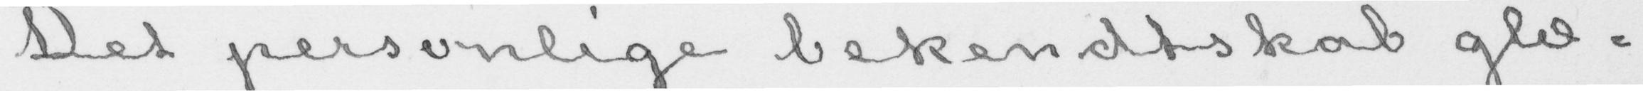Det personlige bekendtskab glæ-
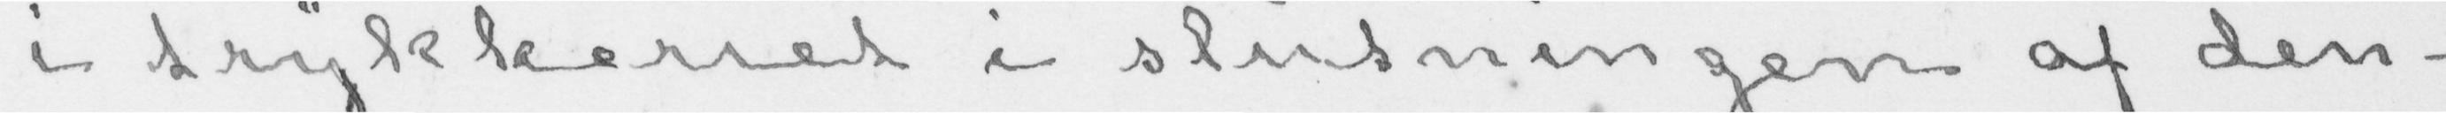i trykkeriet i slutningen af den-
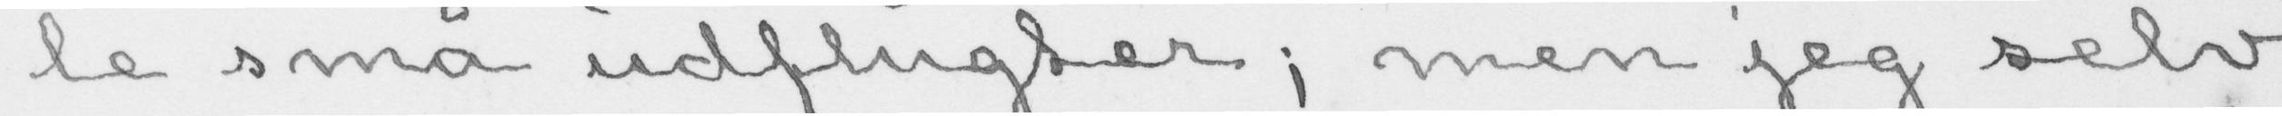le små udflugter; men jeg selv
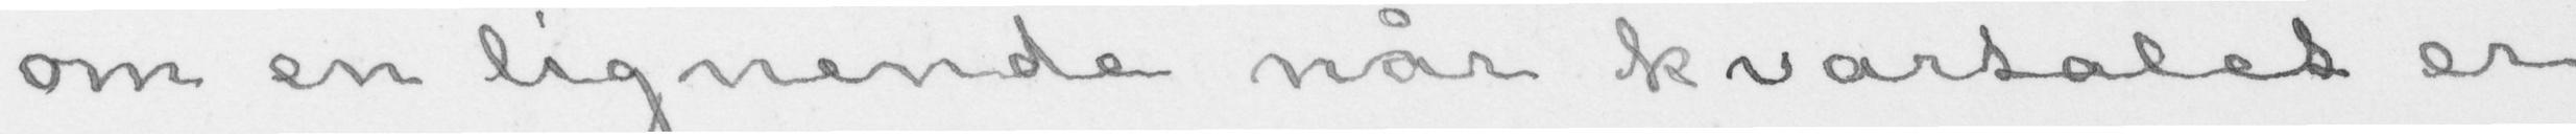om en lignende når kvartalet er
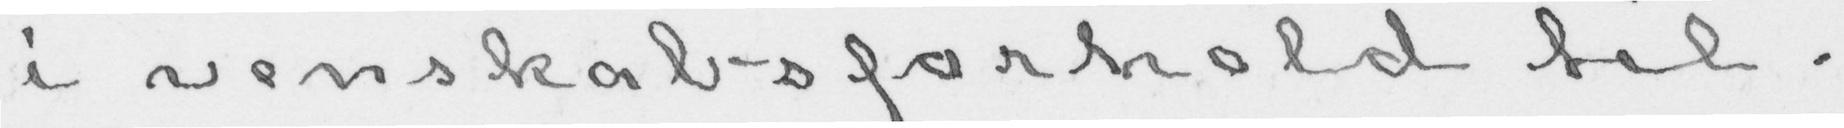i venskabsforhold til.
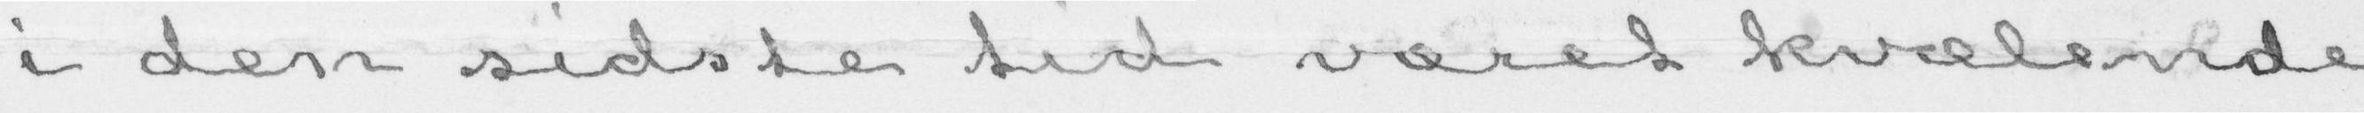i den sidste tid været kvælende
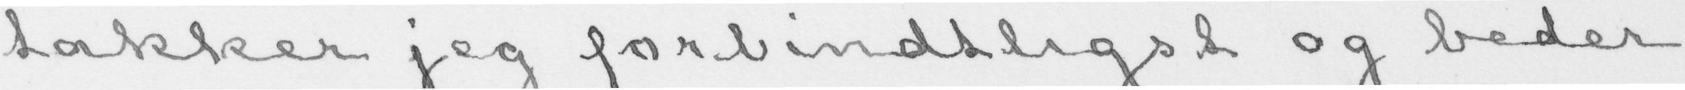takker jeg forbindtligst og beder
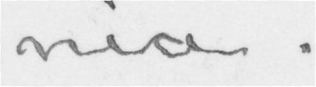nia.-
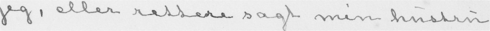jeg, eller rettere sagt min hustru,
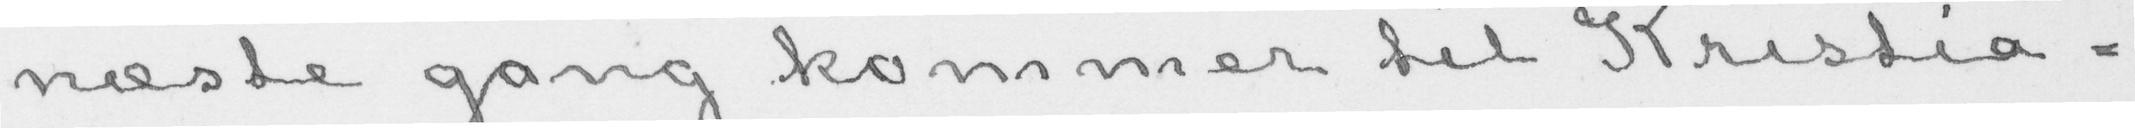næste gang kommer til Kristia-
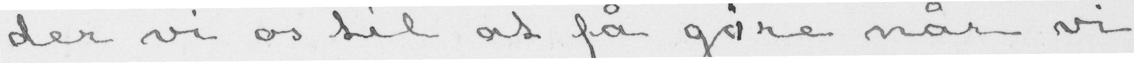der vi os til at få gøre når vi
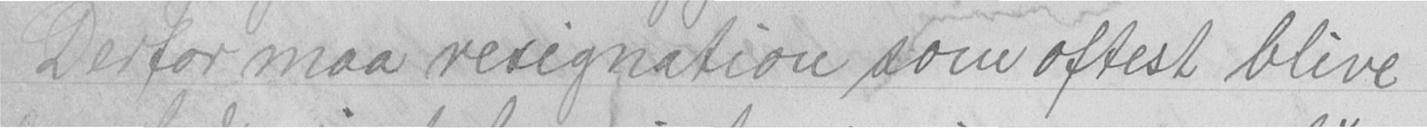Derfor maa resignation som oftest blive
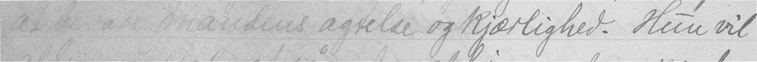at bevare mandens agtelse og kjærlighed. Hun vil
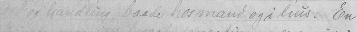ord og handling, baade hos mand og i hus. En
